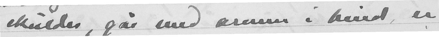skulder, går med armen i bånd, er
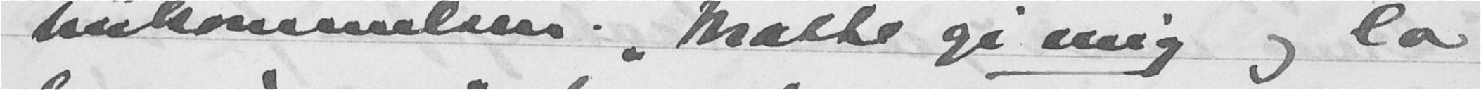hukommelsen. "Måtte gi mig og la
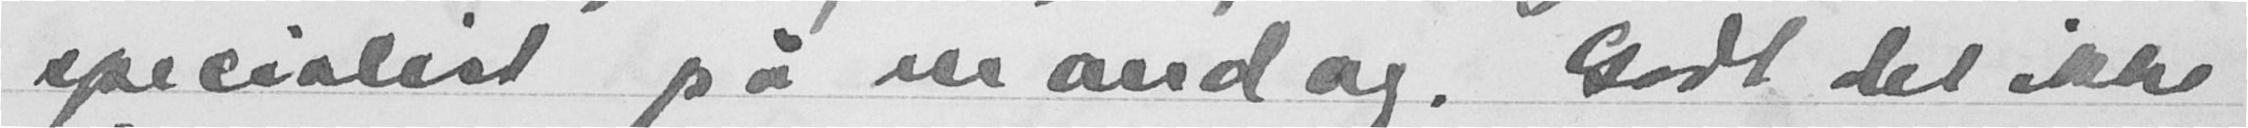specialist på mandag. Godt det ikke
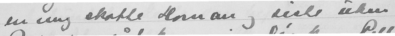en ung skotte Hornan og siste uken
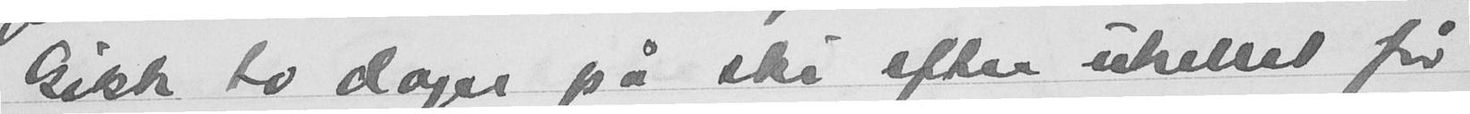Gikk to dager på ski efter uhellet før
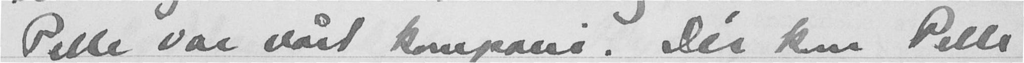Pelle var vårt kompani. Dér kom Pelle
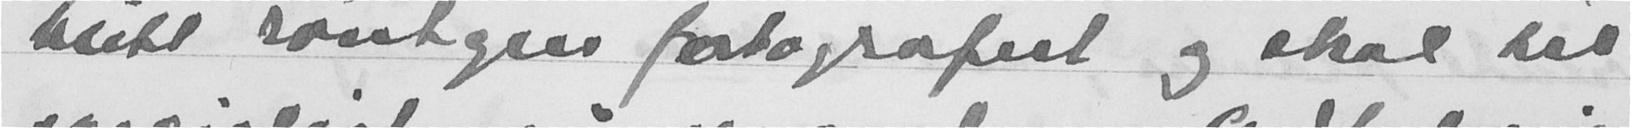blitt røntgen fotografert og skal til
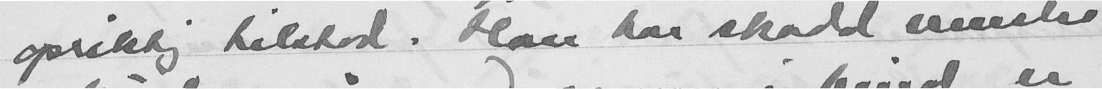opriktig tilstod. Han har skadd venstre
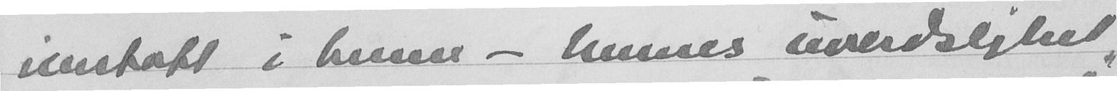inntatt i henne - hennes uverdighet
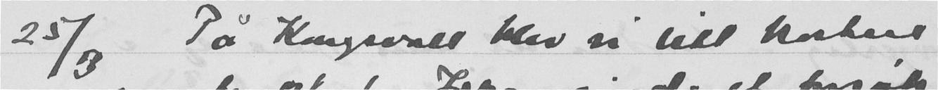28/3 På Kongsvoll blev vi litt kortere
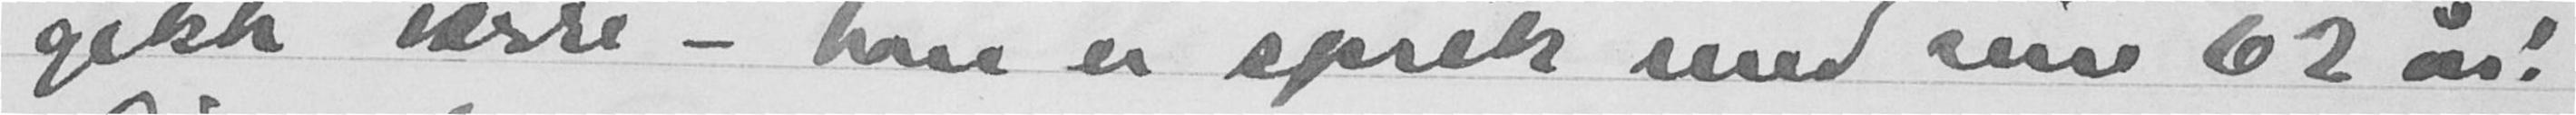gikk værre - han er sprik med sine 62 år!
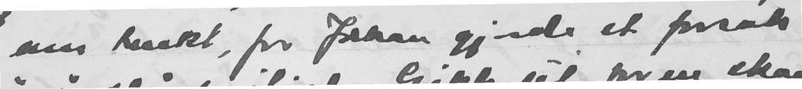enn tenkt, for Johan gjorde et forsøk
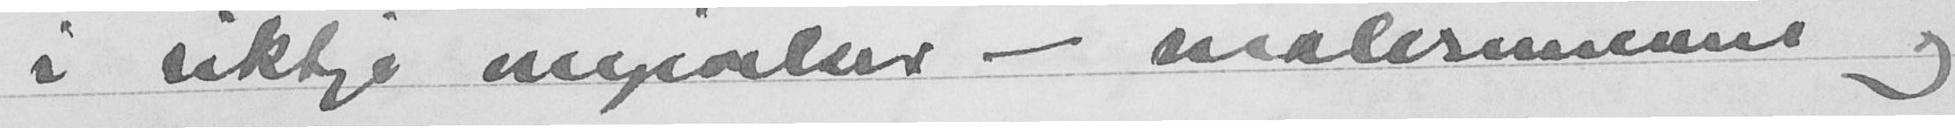i riktige omgivelser - maleriuens og
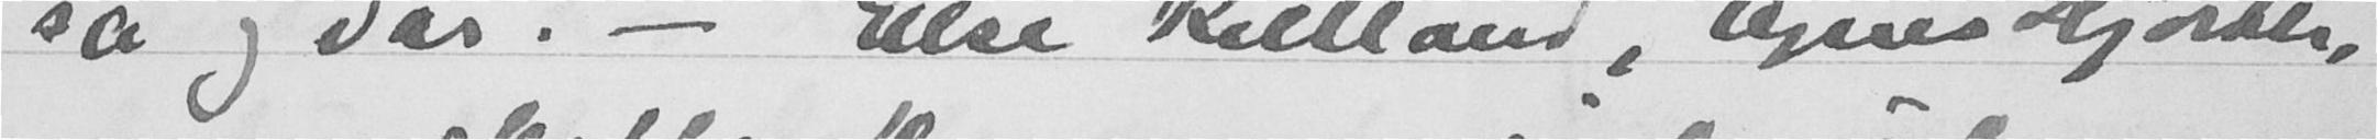så og var. - Else Kielland, Agnes Hjorth,
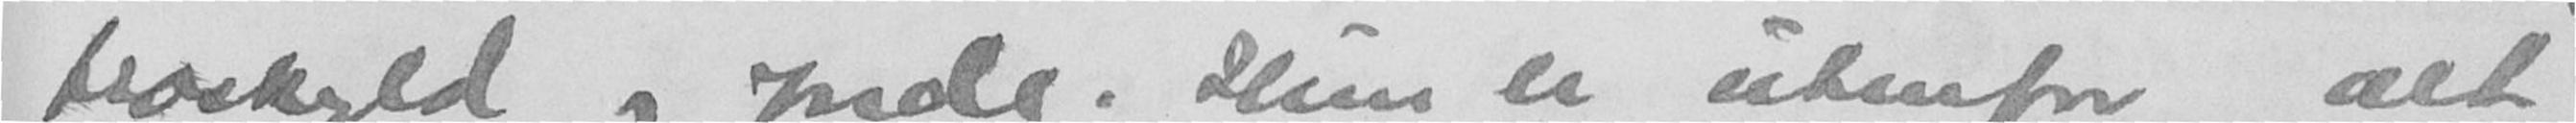troskyld og ynde. Hun er utenfor alt
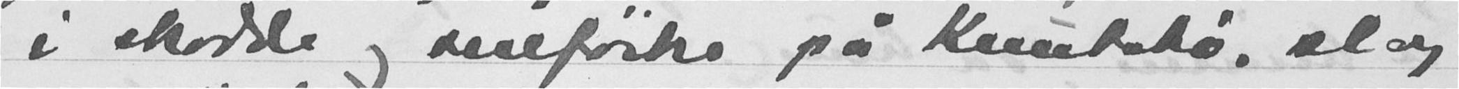i skodde og sneføre på Kunbsbo: slog
